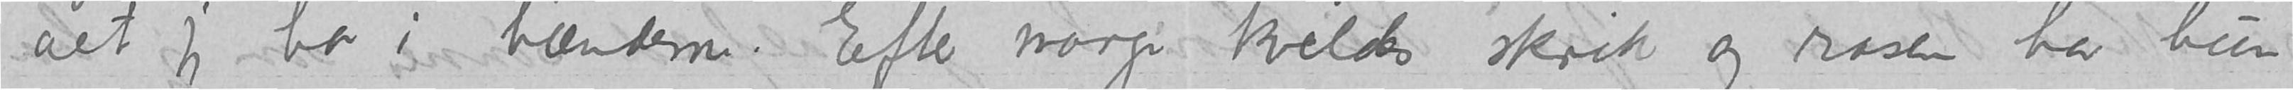alt jeg har i hænderne. Efter mange kveldes skrik og rasen har hun
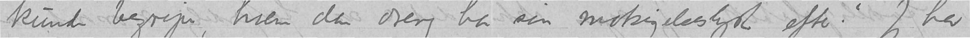kunde begripe, hvem den dreng har sin motsigelseslyst efter.» Jeg har
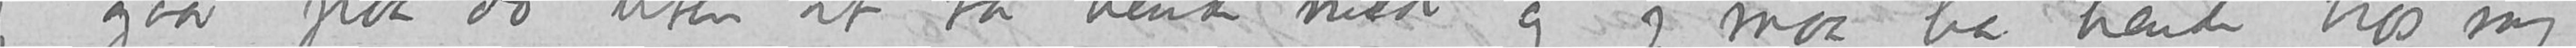jeg gaar paa do uten at ta hende med og jeg maa ha hende hos mig
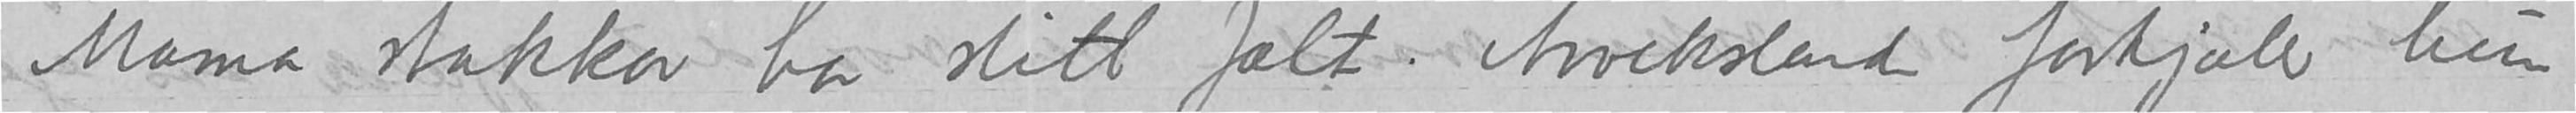Mama stakkar har slitt fælt. Avvekslende forkjæler hun
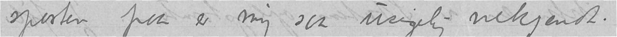sporten paa er mig saa usigelig velkjendt.
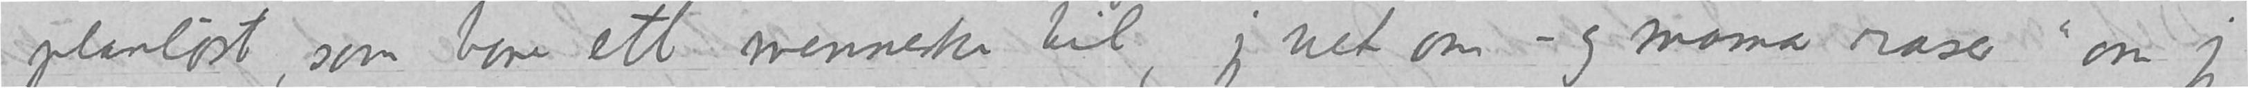planløst, som bare ett menneske til, jeg vet om – og mama raser «om jeg
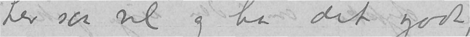Lev saa vel og ha det godt,
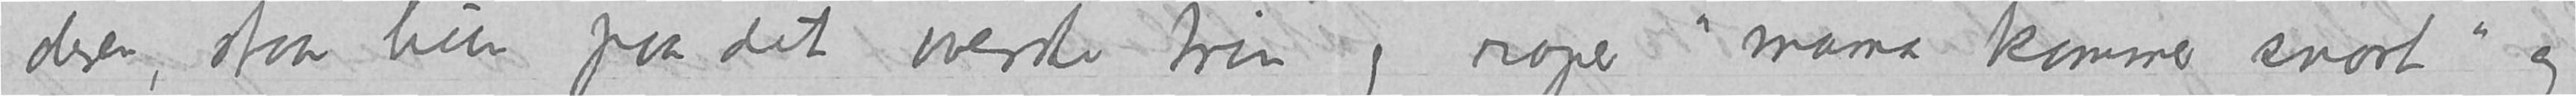deren, staar hun paa det øverste trin og roper «mama kommer snart» og
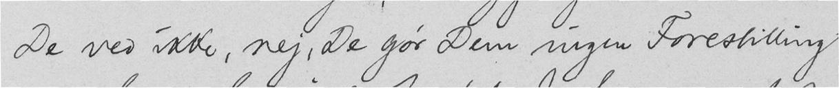De ved ikke, nej, De gør Dem ingen Forestilling
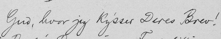Gud, hvor jeg kysser Deres Brev!
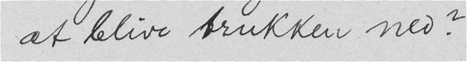at blive trukken ned?
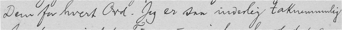Dem for hvert Ord. Jeg er saa inderlig taknemmelig
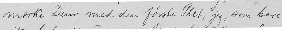mærke Dem med den første Plet, jeg, som bare
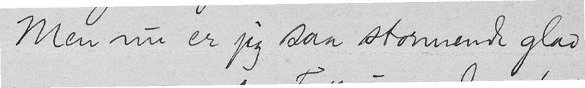Men nu er jeg saa stormende glad
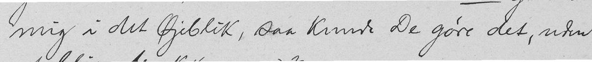mig i det Øjeblik, saa kunde De gøre det, uden
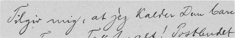Tilgiv mig, at jeg kalder Dem bare
